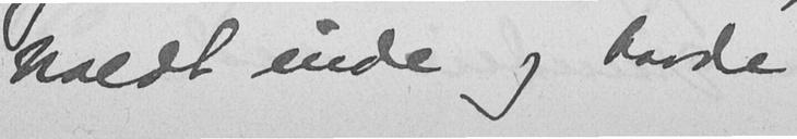holdt inde og havde
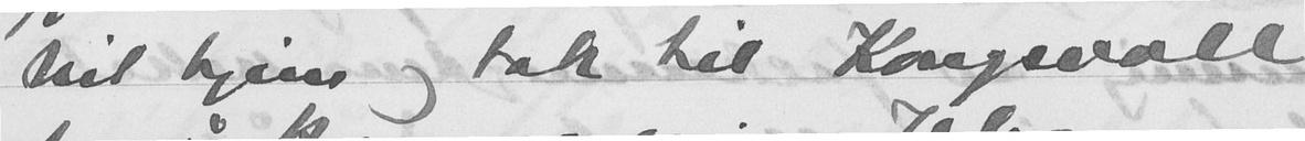hit hjem og tok til Kongsvoll
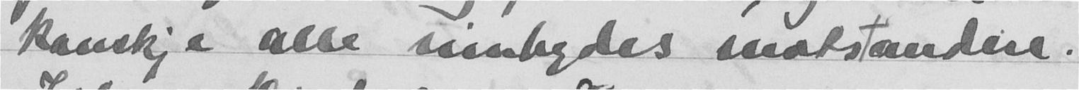kanskje alle innbyrdes motstandere.
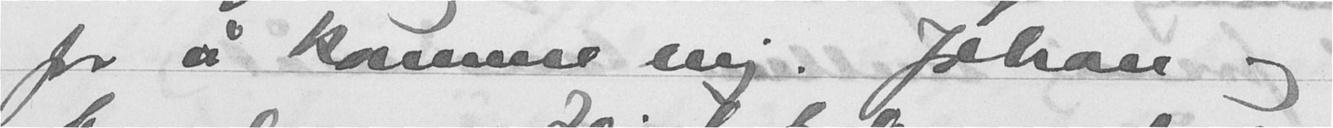for å komme mig. Johan og
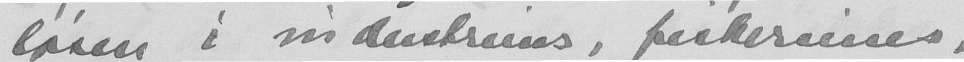læsen i in dustrums, piskessesses,
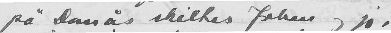på Domås skiltes Johan og jeg,
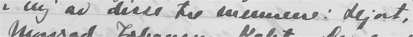i mig av disse tre mennene: Hjort,
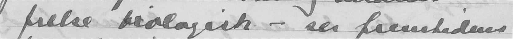frelse biologisk - ser fremtidens
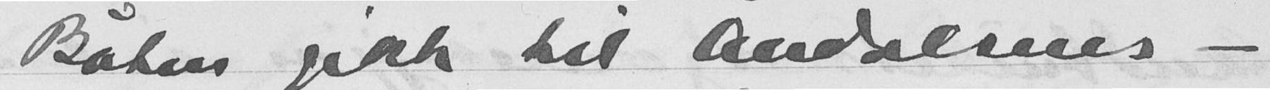Båten gikk til Åndalsens -
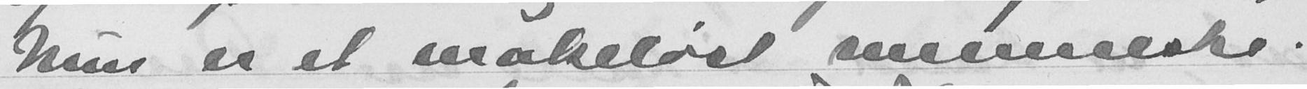Hun er et makeløst menneske.
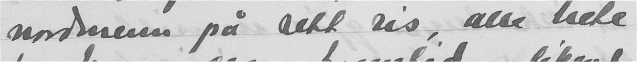nordmenn på rett vis, alle levde
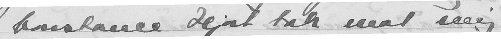Constance Hjort tok imot mig
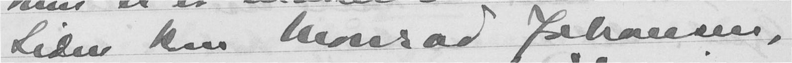Siden kom Monrad Johansen,
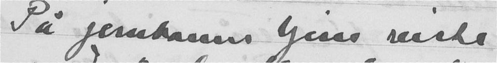På jernbanen hjem reiste
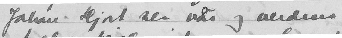Johan Hjort ser vår og verdens
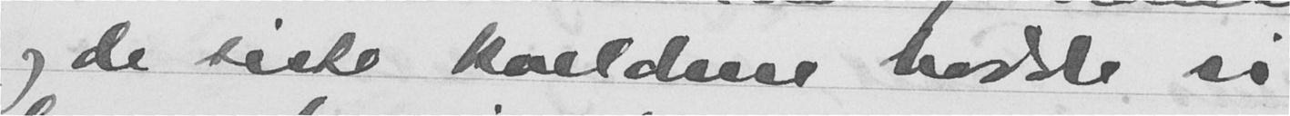og de siste kveldene hadde vi
